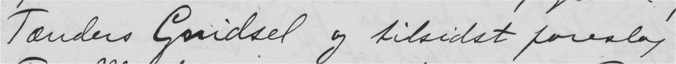Tænders Gnidsel og tilsidst foreslog
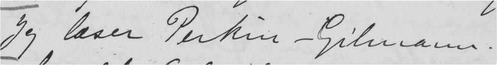Jeg læser Perkin-Gilmann.
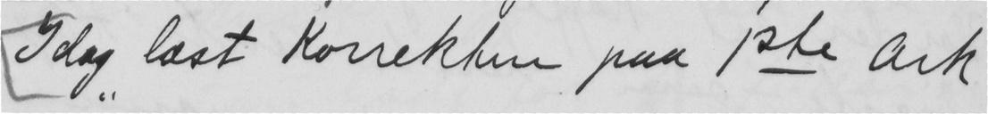Idag læst Korrektur paa 1ste Ark
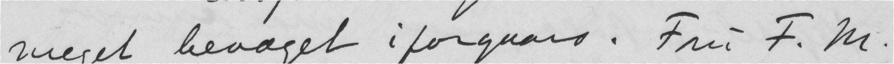meget bevæget iforgaars. Fru F. M.
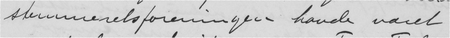stemmeretsforeningen havde været
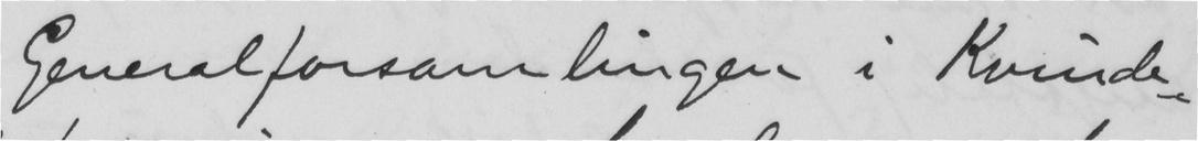Generalforsamlingen i Kvinde-
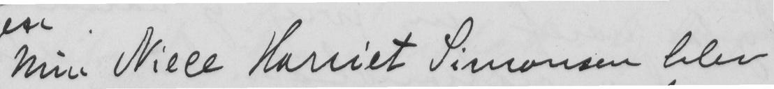min Niece Harriet Simonsen blev
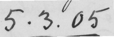5.3.05
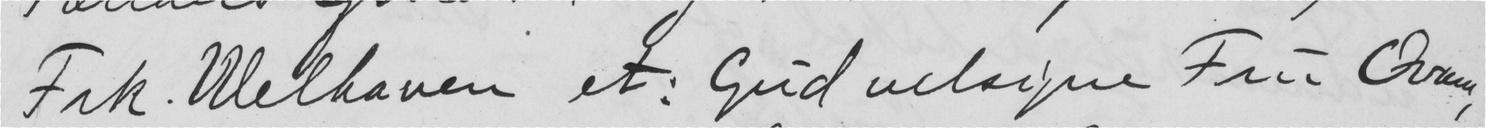Frk Welhaven et: Gud velsyne Fru Qvam,
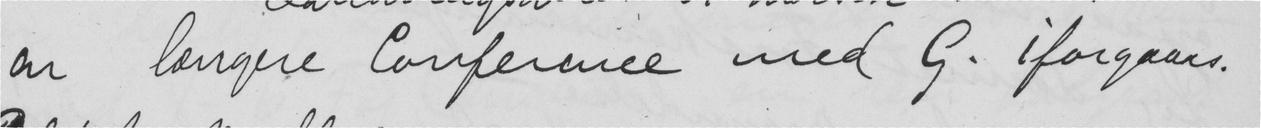en længere Conference med G. iforgaars.
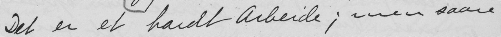Det er et hardt Arbeide; men saare
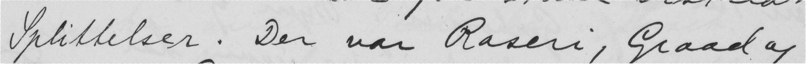Splittelser. Der var Raseri, Graad og
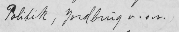Politik, Jordbrug o.s.v.
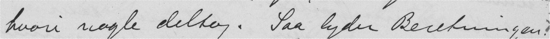hvori nogle deltog. Saa lyder Beretningen.
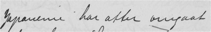Japanerne har atter omgaat
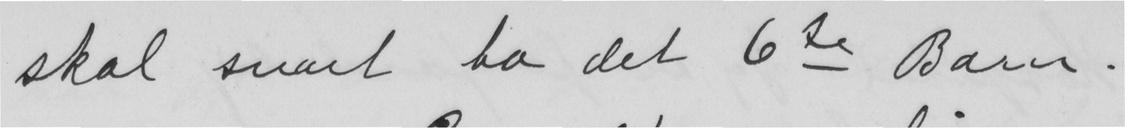skal snart ha det 6te Barn.
